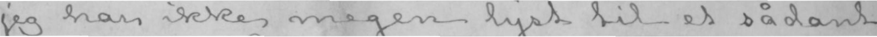jeg har ikke megen lyst til et sådant
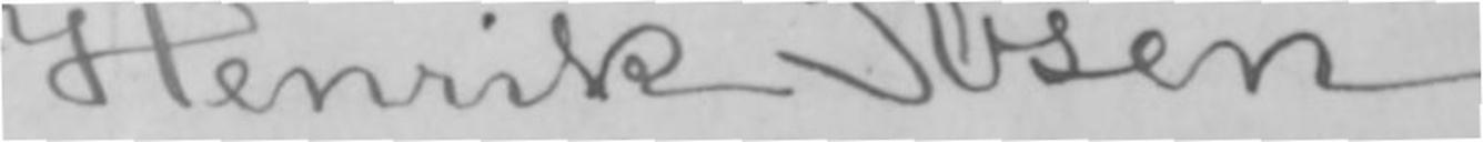Henrik Ibsen.
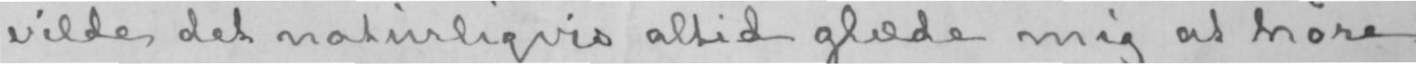vilde det naturligvis altid glæde mig at høre
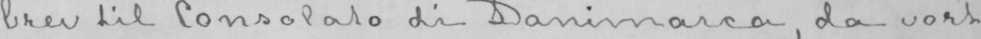brev til Consolato di Danimarca, da vort
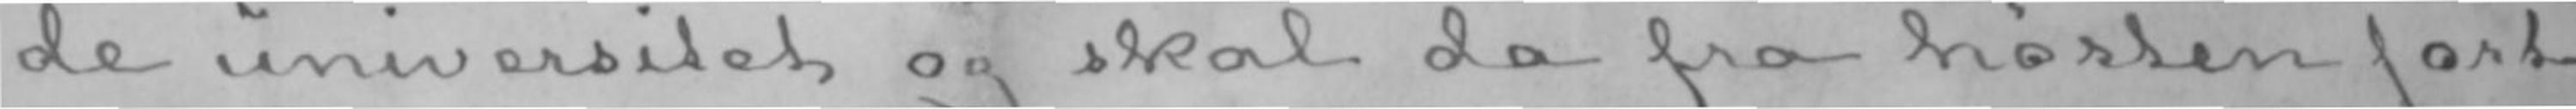de universitet og skal da fra høsten fort-
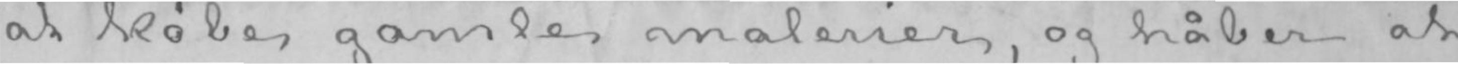at købe gamle malerier, og håber at
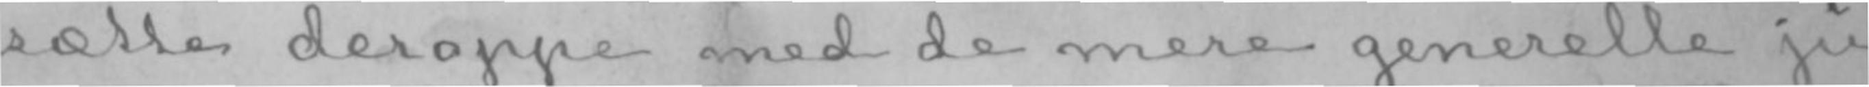sætte deroppe med de mere generelle ju-
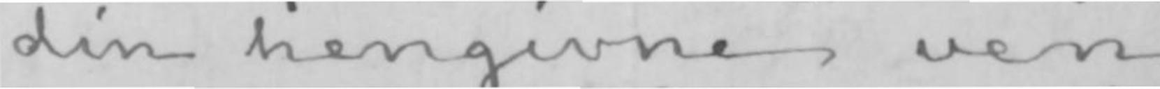din hengivne ven
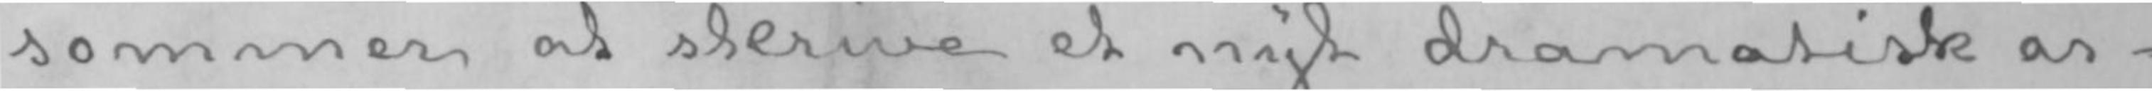sommer at skrive et nyt dramatisk ar-
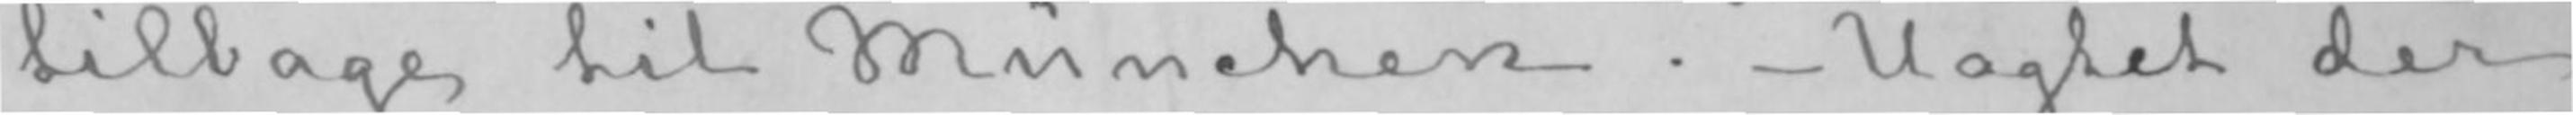tilbage til München.- Uagtet der
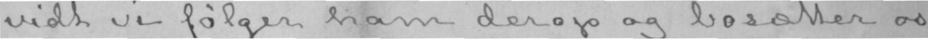vidt vi følger ham derop og bosætter os
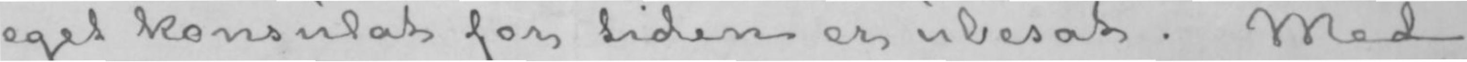eget konsulat for tiden er ubesat. Med
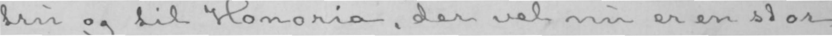tru og til Honoria, der vel nu er en stor
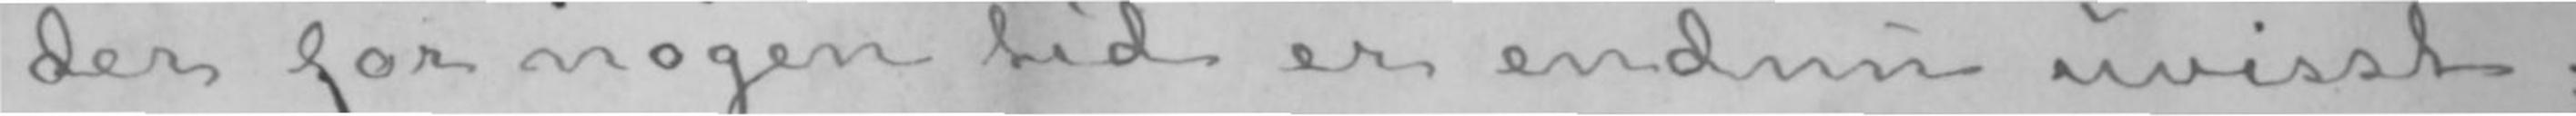der for nogen tid er endnu uvisst;
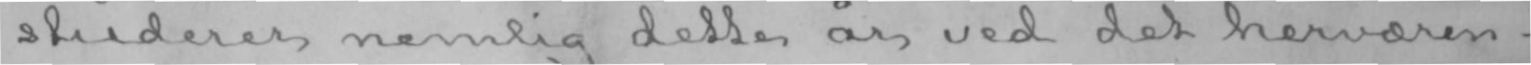studerer nemlig dette år ved det herværen-
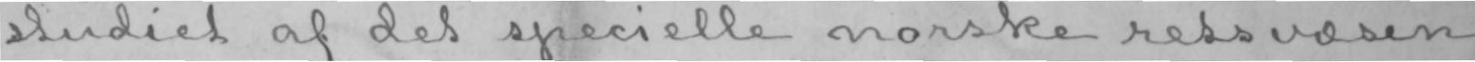studiet af det specielle norske retsvæsen
